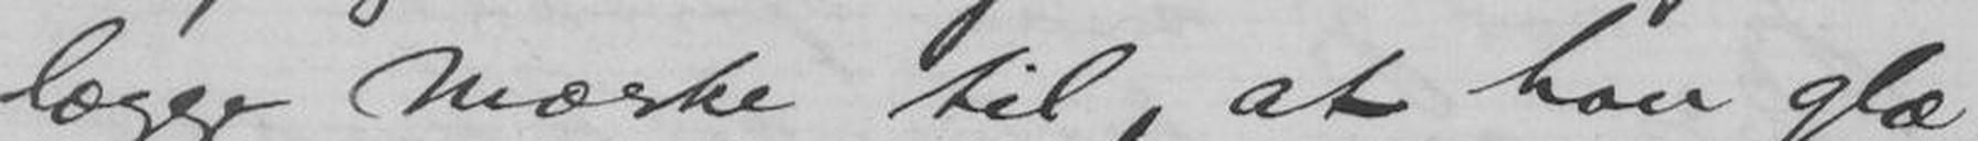lægge mærke til, at han glæ-
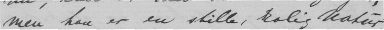men han er en stille, rolig Natur,
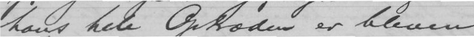hans hele Optræden er bleven
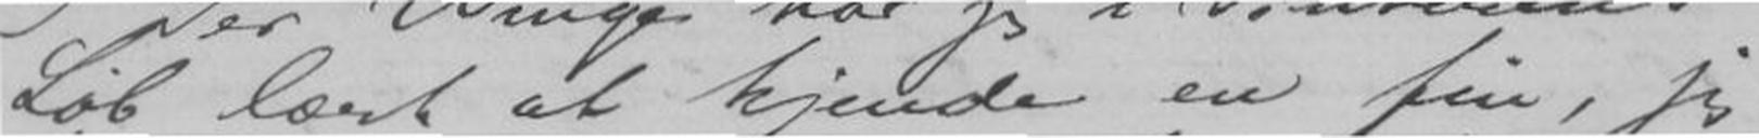Løb lært at kjende en fin, jeg
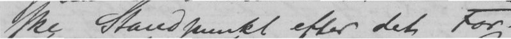ske Standpunkt efter det For-
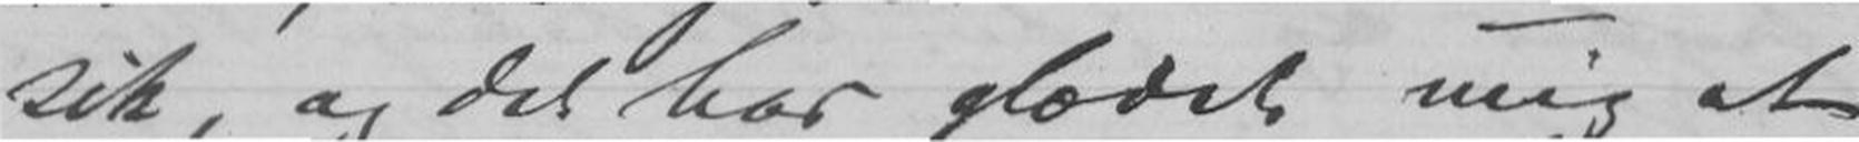sik, og det har glædet mig at
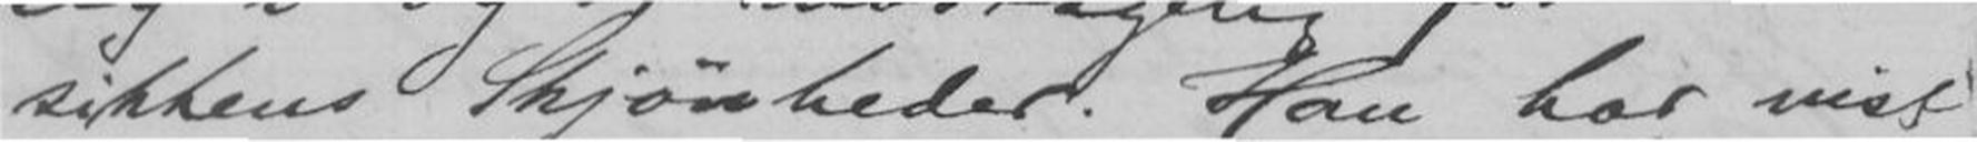sikkens Skjønheder. Han har vist-
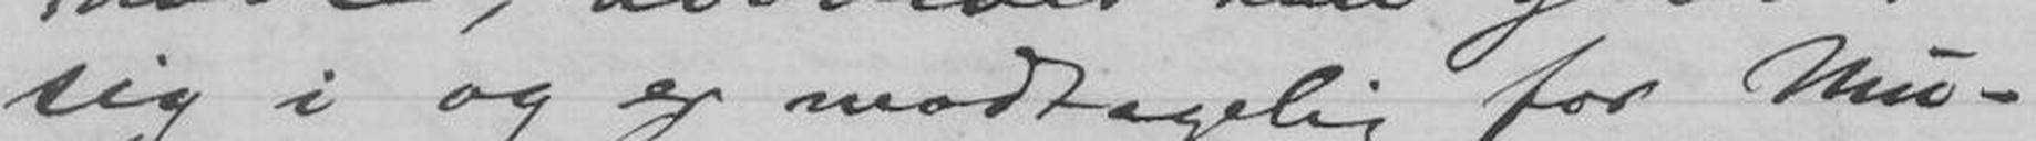sig i og er modtagelig for Mu-
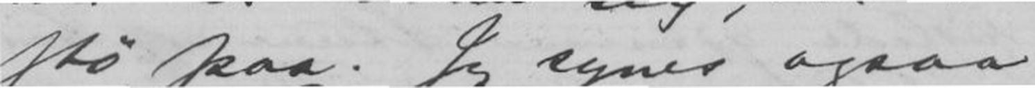jeg stø paa. Jeg synes ogsaa
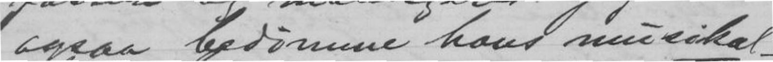ogsaa bedømme hans musikal-
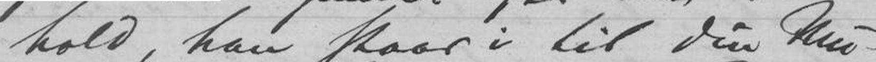hold, han staar i til Din Mu-
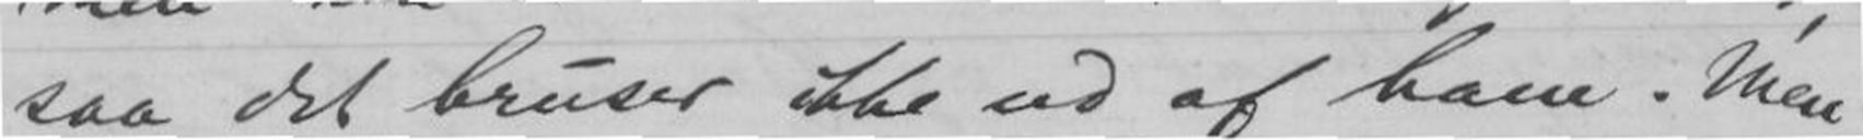saa det bruser ikke ud af ham. Men
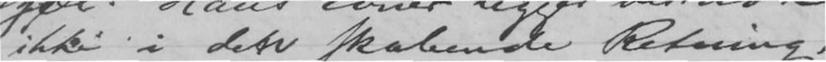ikke i den skabende Retning,
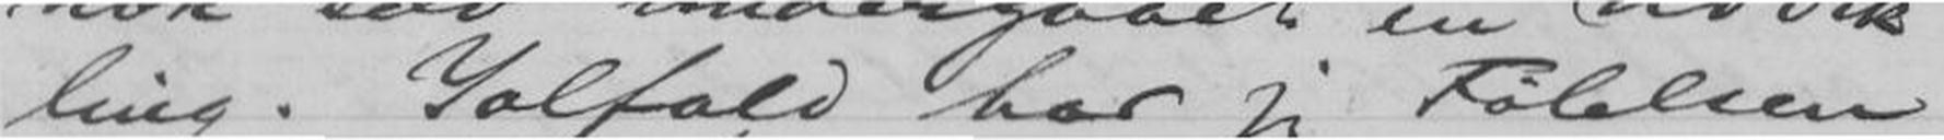ling. Ialtfald har jeg Følelsen
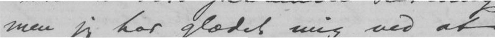men jeg har glædet mig ved at
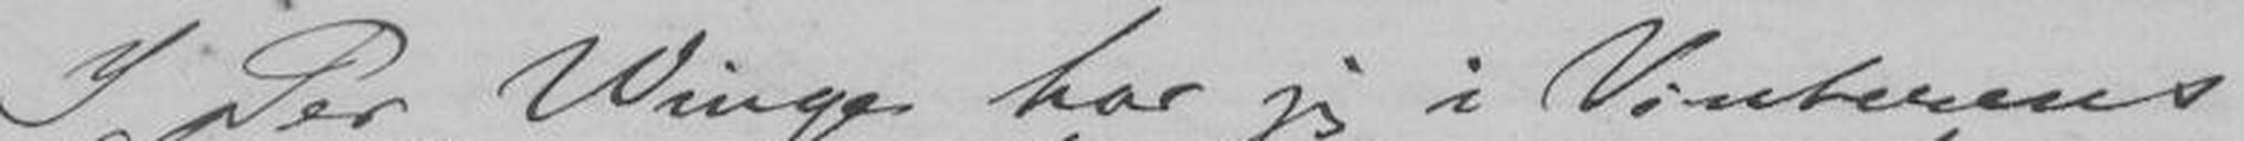I Per Winge har jeg i Vinterens
